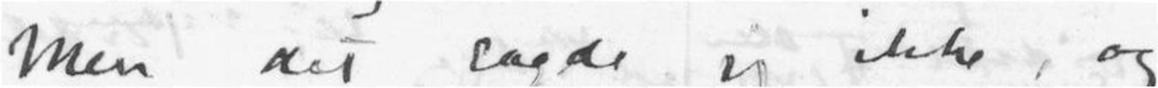Men det sagde jeg ikke, og
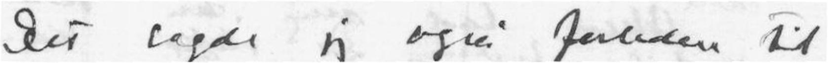Det sagde jeg også forleden til
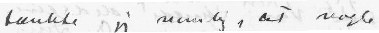tænkte jeg nemlig, at nogle
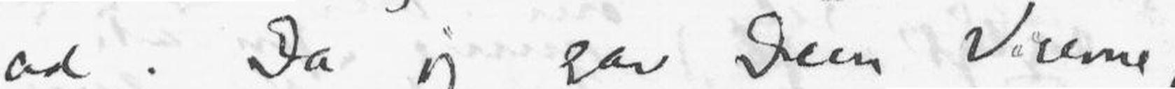ad. Da jeg gav Dem Viserne,
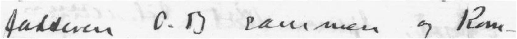fatteren O. B. sammen og kom-
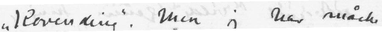Kovending. Men jeg har måske
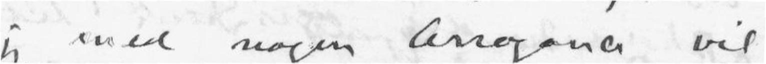jeg med nogen Arrogance vil
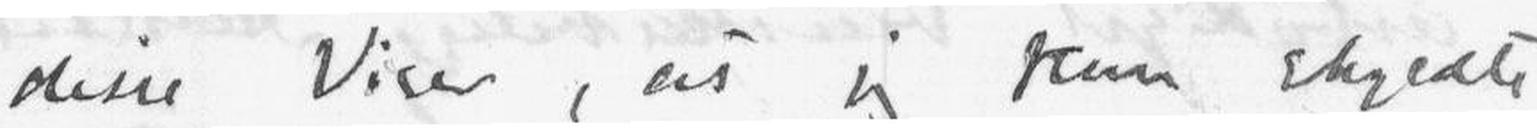disse Viser, at jeg kun skyldte
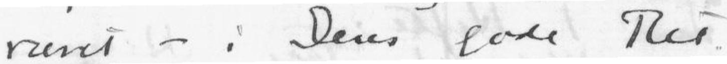været - i Deres gode Ret.
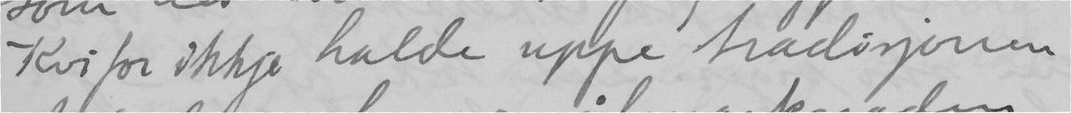Kvifor ikkje halde uppe tradisjonen
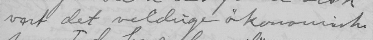vort det veldige ökonomiske
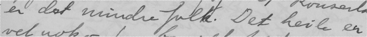er det mindre folk. Det heile er
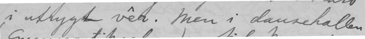i utryggt vær. Men i dansehallen
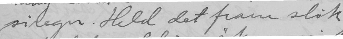siregn. Held det fram slik
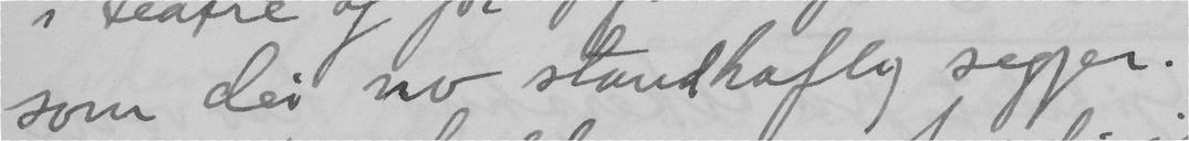som dei no standhaftig segjer.
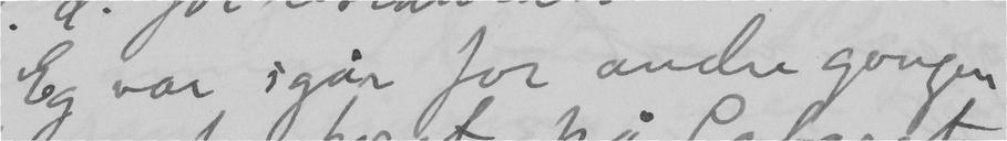Eg var igår for andre gongen
